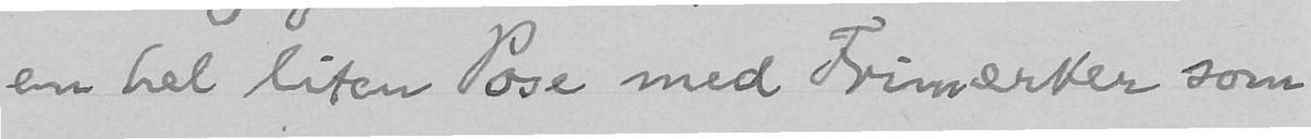en hel liten Pose med Frimærker som
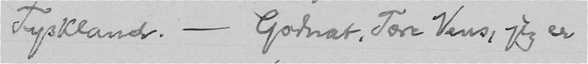Tyskland. - Godnat, Tore Vens, jeg er
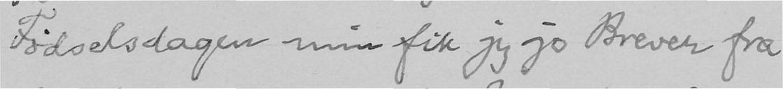Fødselsdagen min fik jeg jo Brever fra
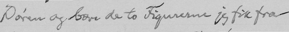Døren og bære de to Figurerne jeg fik fra
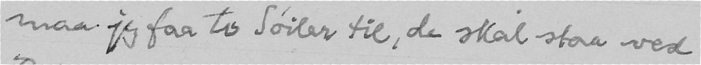maa jeg faa to Søiler til, de skal staa ved
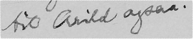til Arild ogsaa.
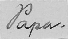Papa.
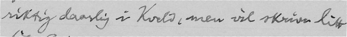riktig daarlig i Kveld, men vil skrive litt
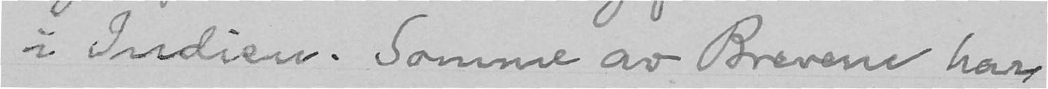i Indien. Somme av Brevene har
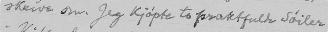skrive om. Jeg kjøpte to praktfulde Søiler
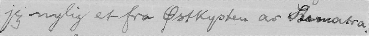jeg nylig et fra Østkysten av Sumatra.
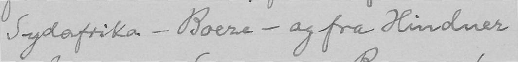Sydafrika - Boere - og fra Hinduer
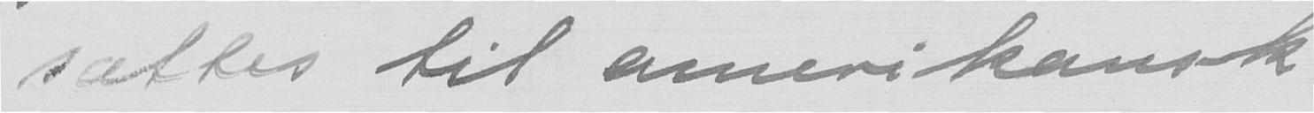sættes til amerikansk
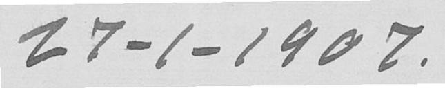27-1-1907.
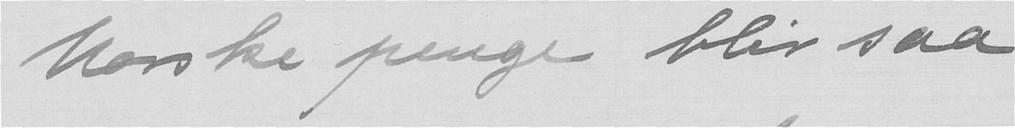Norske penge blir saa
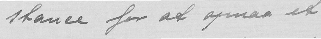stance for at opnaa et
